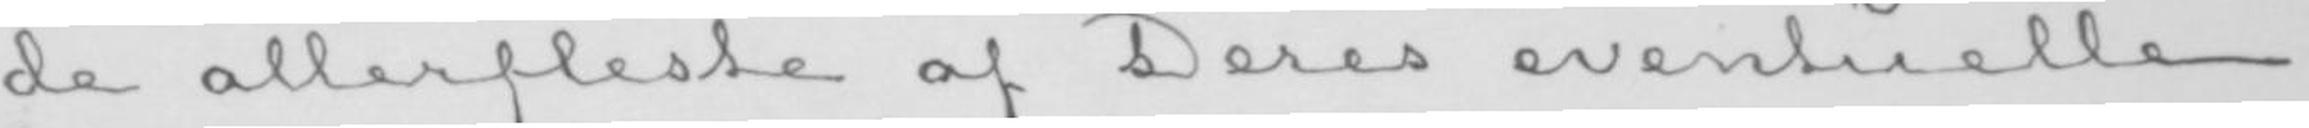de allerfleste af Deres eventuelle
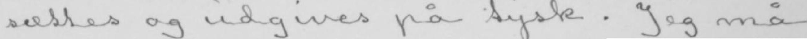sættes og udgives på tysk. Jeg må
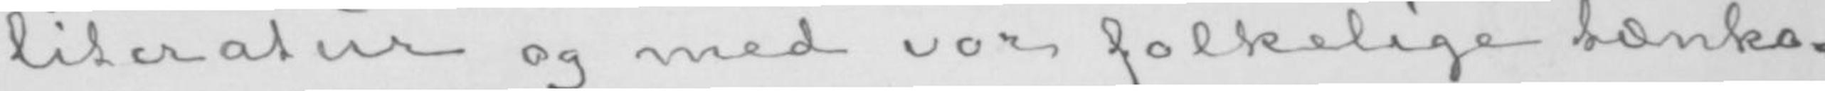literatur og med vor folkelige tænke-
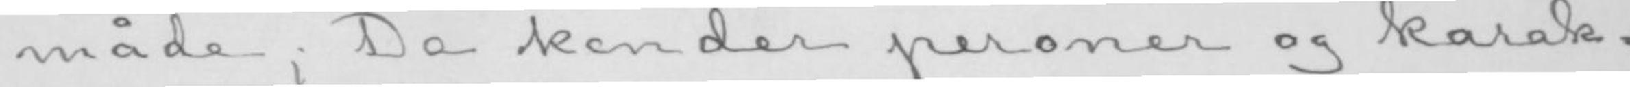måde; De kender peroner og karak-
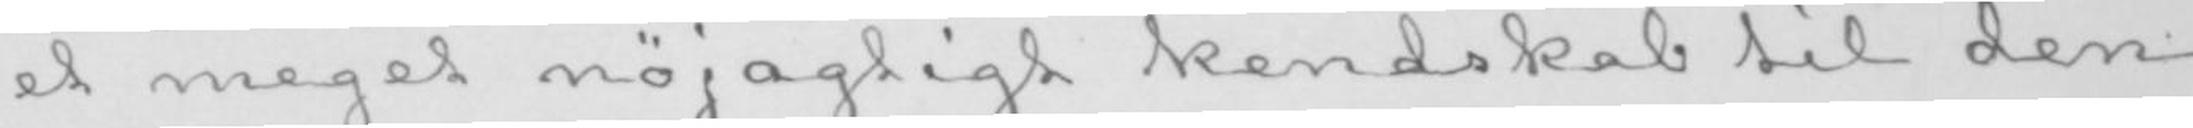et meget nøjagtigt kendskab til den
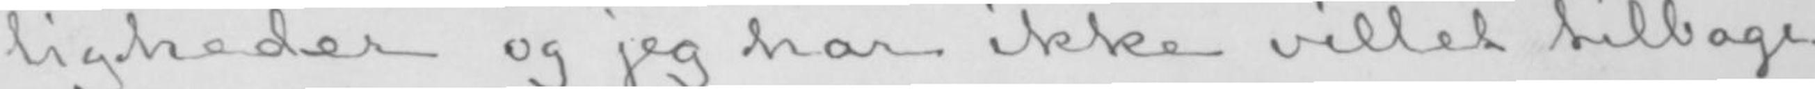ligheder og jeg har ikke villet tilbage-
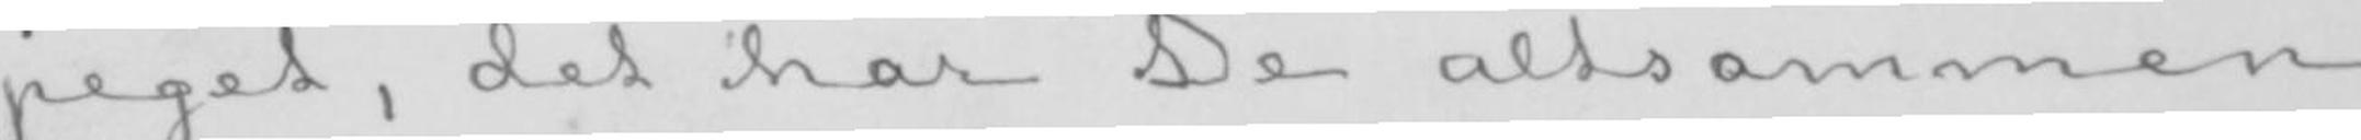peget, det har De altsammen
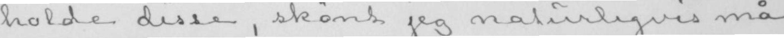holde disse, skønt jeg naturligvis må
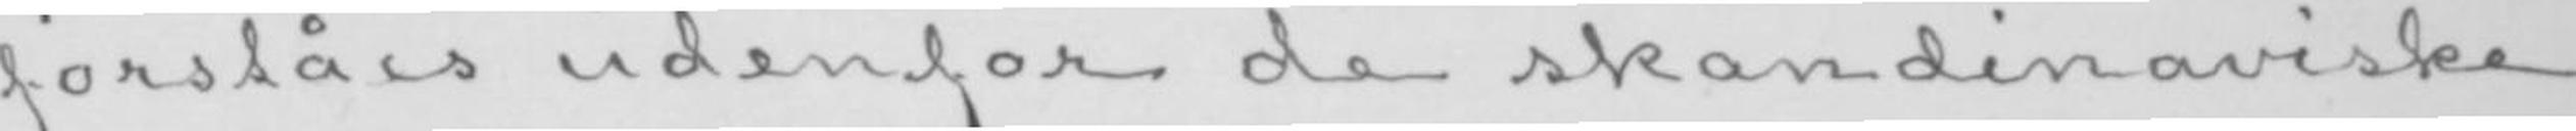forståes udenfor de skandinaviske
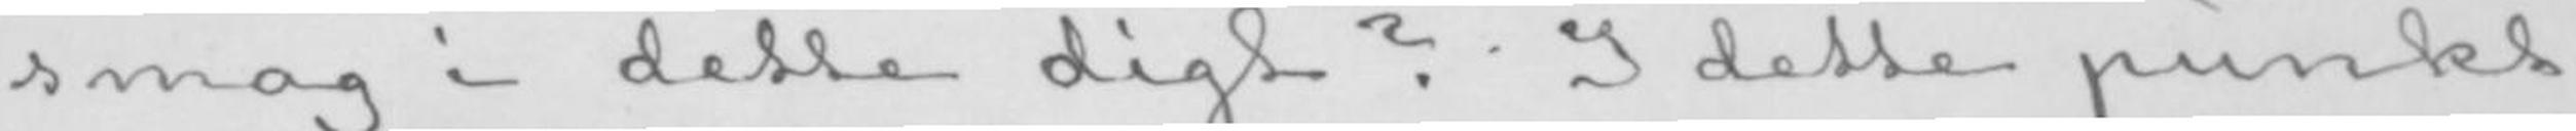smag i dette digt? I dette punkt
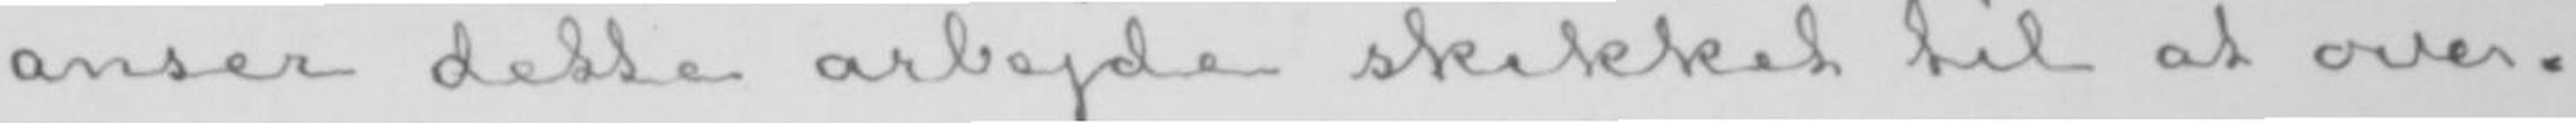anser dette arbejde skikket til at over-
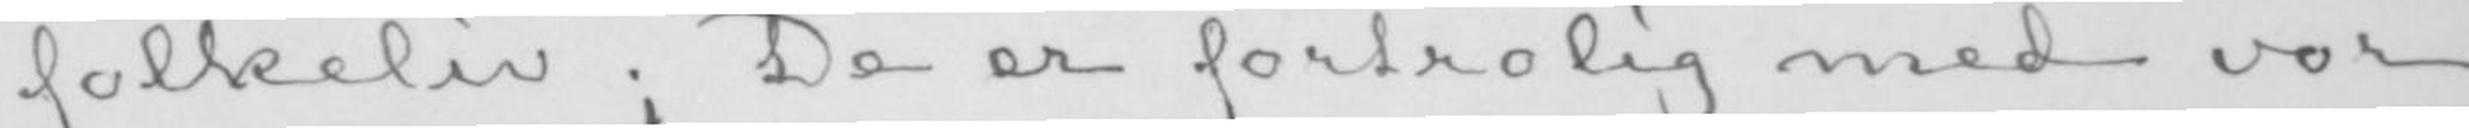folkeliv; De er fortrolig med vor
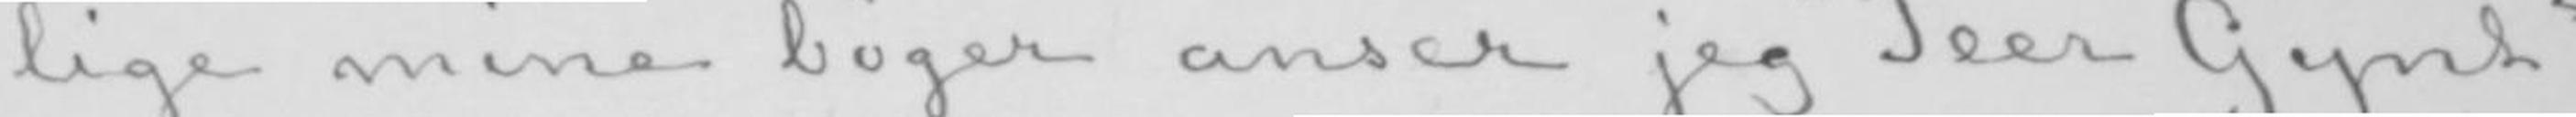lige mine bøger anser jeg «Peer Gynt»
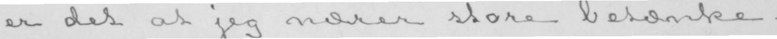er det at jeg nærer store betænke-
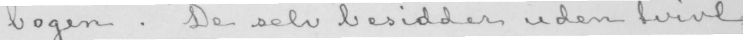bogen. De selv besidder uden tvivl
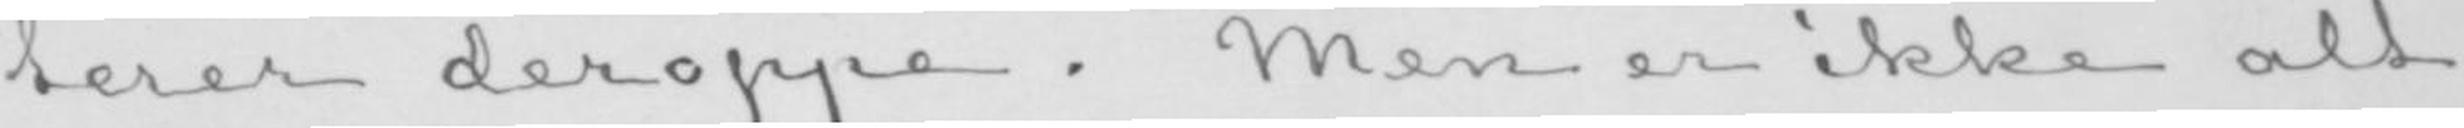terer deroppe. Men er ikke alt
